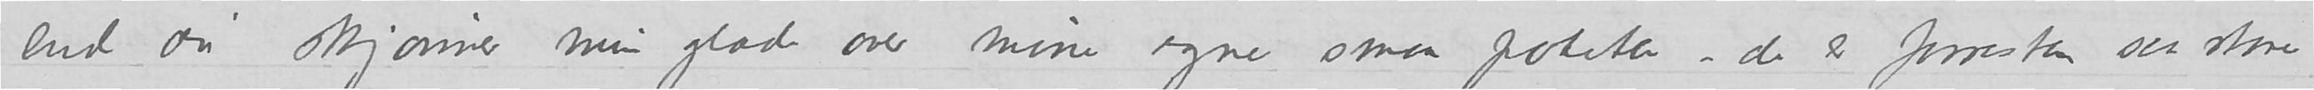end du skjønner min glæde over mine egne smaa poteter - de er forresten saa store
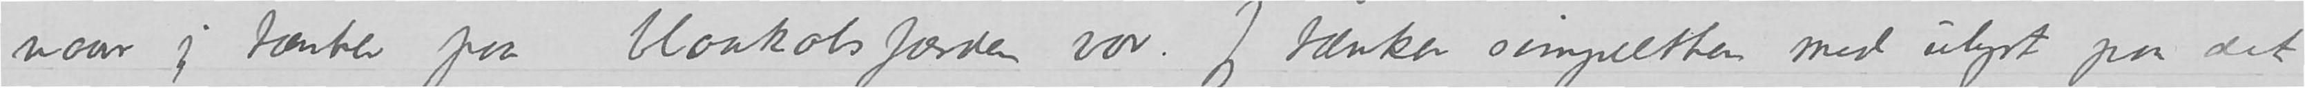naar jeg tænker paa blaakolsferden vor. Jeg tænker simpelthen med ulyst paa det
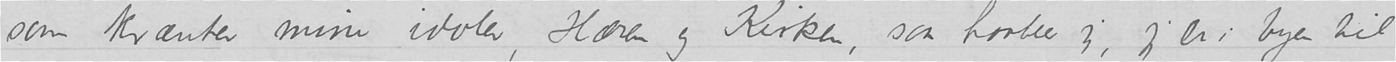som krænker mine idoler, Hæren og Kirken, saa haaber jeg, jeg er i byen til
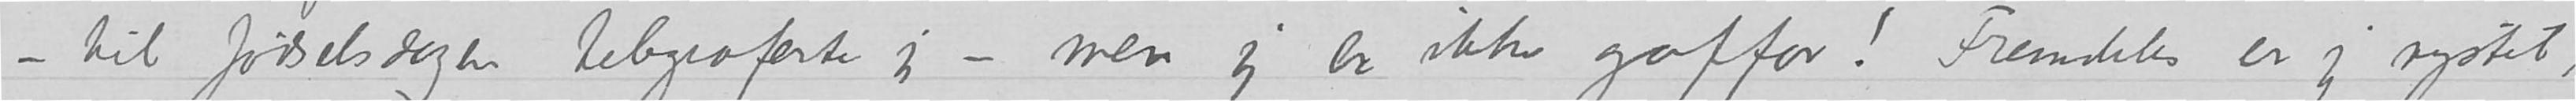- til fødselsdagen telegraferte jeg - men jeg er ikke gaffar! Fremdeles er jeg rystet,
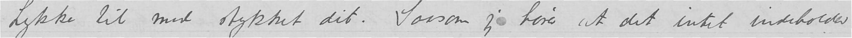Lykke til med stykket dit. Saasom jeg hører at det intet indeholder
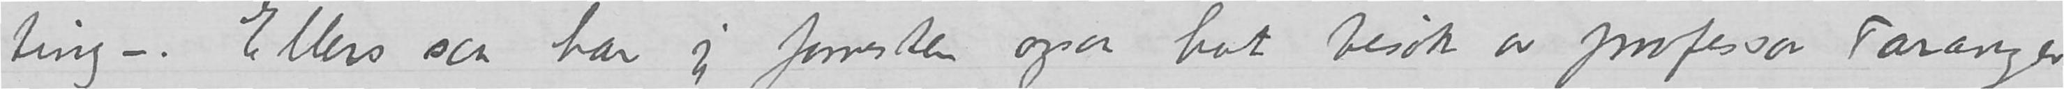ting -. Ellers saa har jeg forresten ogsaa havt besøk av professor Taranger
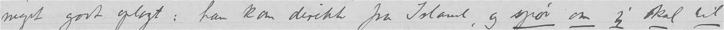meget godt oplagt: han kom direkte fra Island, og spør om jeg skal til
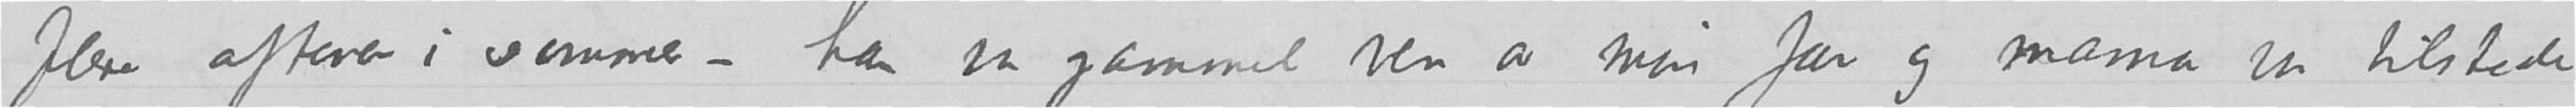flere aftener i sommer - han var gammel ven av min Far og mama var tilstede
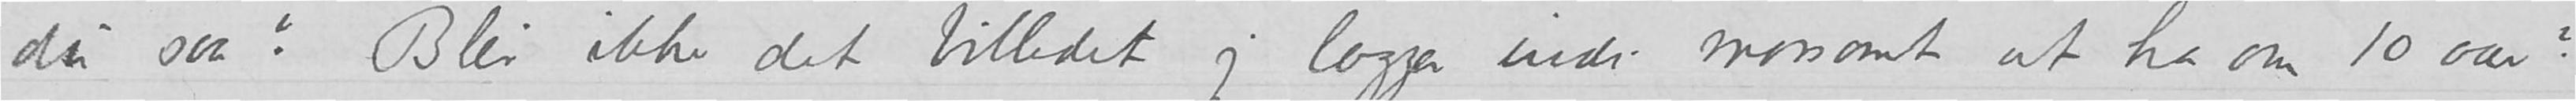du saa! Blir ikke det billedet jeg legger udi morsomt at ha om 10 aar?
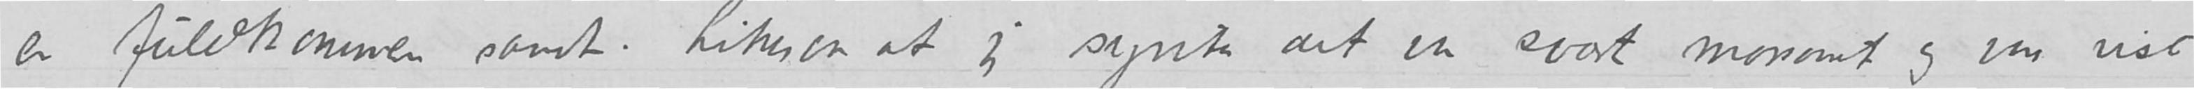er fuldkommen sandt. Likesaa at jeg syntes det var svært morsomt og var vist
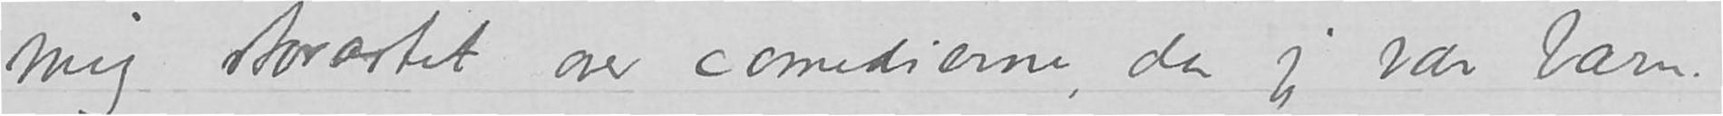meg storartet over comedierne, da jeg var barn.
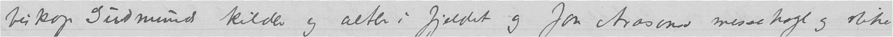biskop Gudmunds kilder og alter i fjeldet og Jon Arosons messehagl og slike
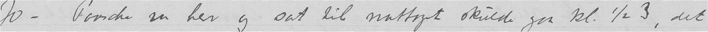Jo - Paasche var her og sat til nattoget skulde gaa kl. ½ 3, det
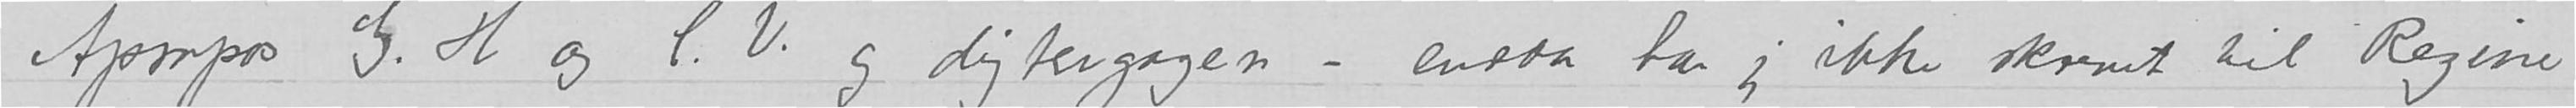Apropos G.H. og C.V. og digtergagen - endda har jeg ikke skrevet til Regine
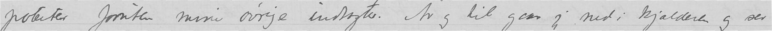poteter foruten mine øvrige indtægter. Av og til gaar jeg ned i kjælderen og ser
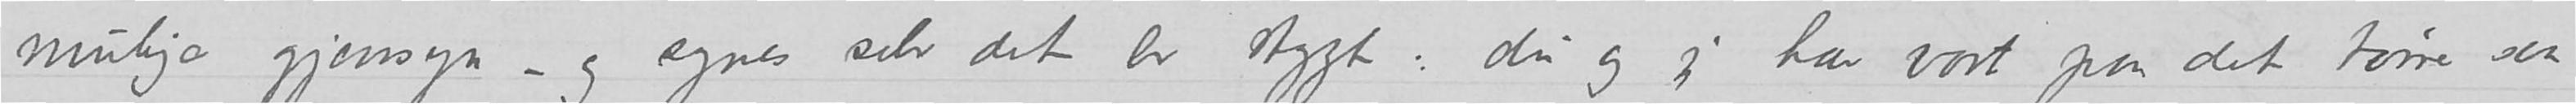mulige gjensyn - og synes selv det er stygt: du og jeg har vort paa det tørre saa
